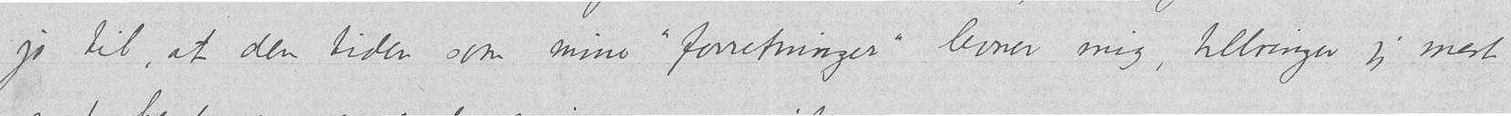jo til, at den tiden som mine "forretninger" levner mig, tilbringer jeg mest
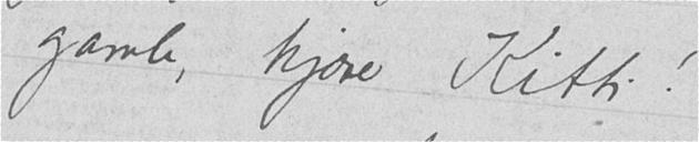gamle, kjære Kitti!
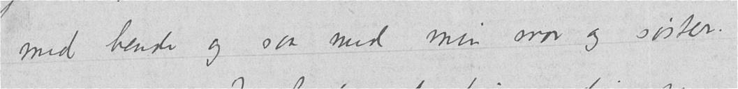med hende og saa med min mor og søster.
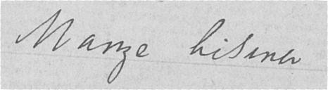Mange hilsener
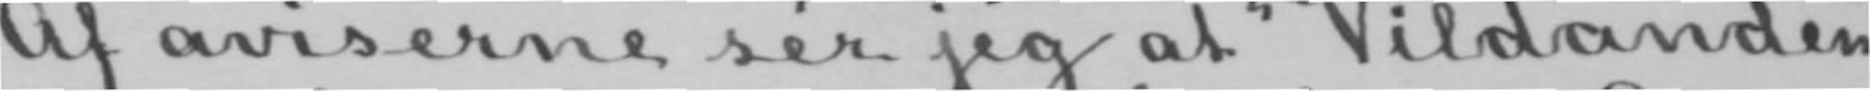Af aviserne sér jeg at «Vildanden»
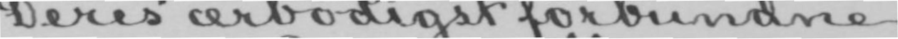Deres ærbødigst forbundne
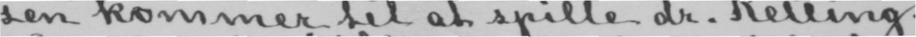sen kommer til at spille dr. Relling.
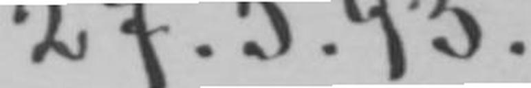27.5.93.
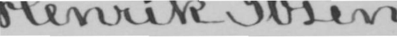Henrik Ibsen.
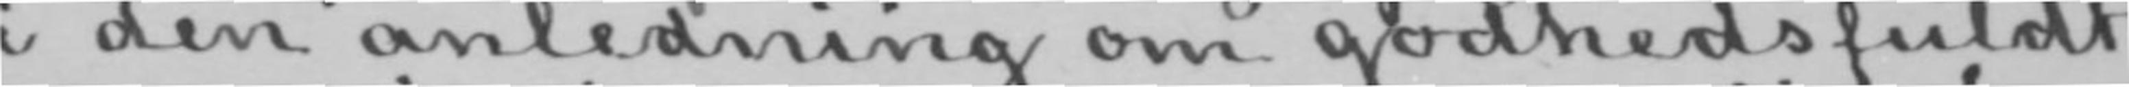i den anledning om godhedsfuldt
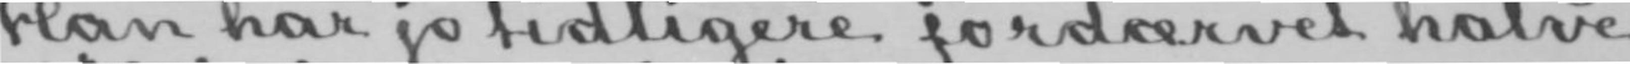Han har jo tidligere fordærvet halve
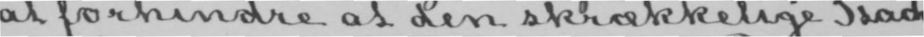at forhindre at den skrækkelige Isach
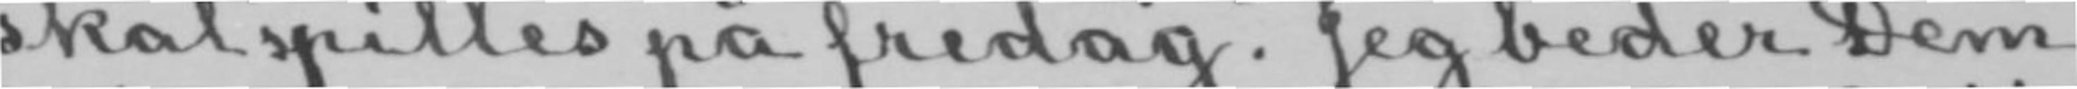skal spilles på fredag. Jeg beder Dem
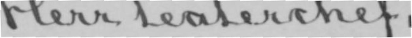Herr teaterchef,
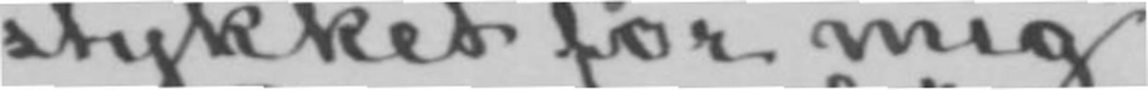stykket for mig!
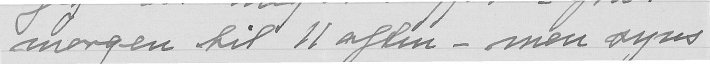morgen til 11 aften - men syns
